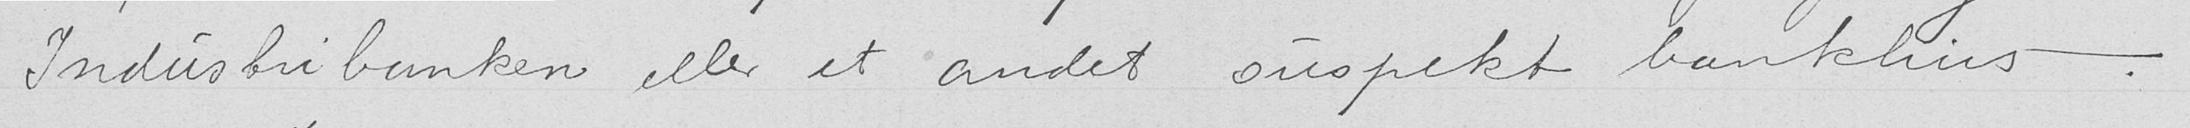Industribanken eller et andet suspekt bankhus -.
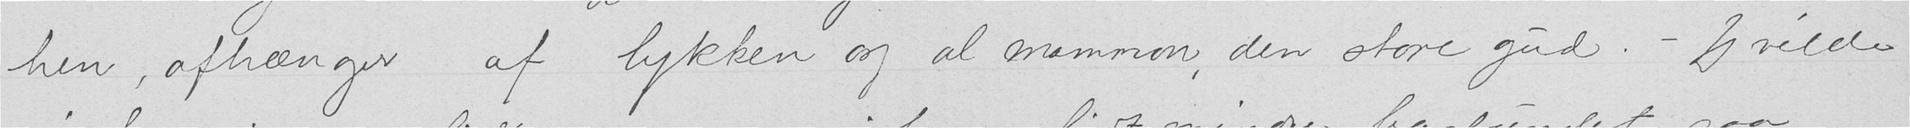hen, afhænger af lykken og af mammon, den store gud. - Jeg vilde
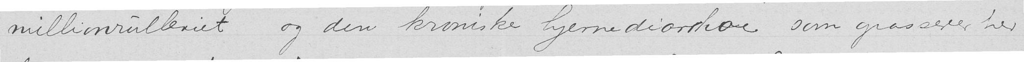millionrulleriet og den kroniske hjernediarrhoe som grasserer her
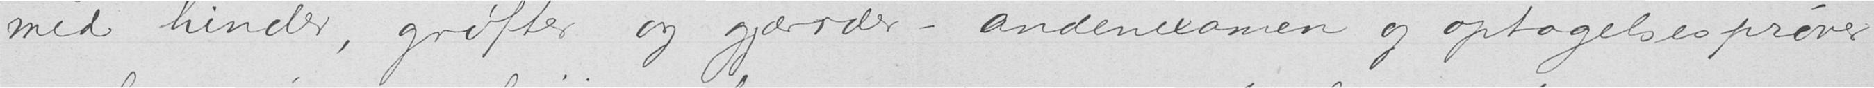med hinder, grøfter og gjærder- andenexamen og optagelsesprøver
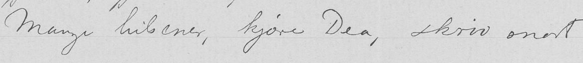Mange hilsener, kjære Dea, skriv snart
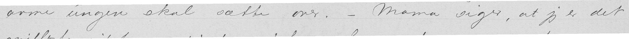arme ungen skal sætte over - Mama siger, at jeg er det
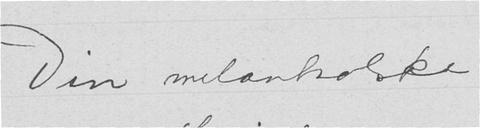Din melankolske
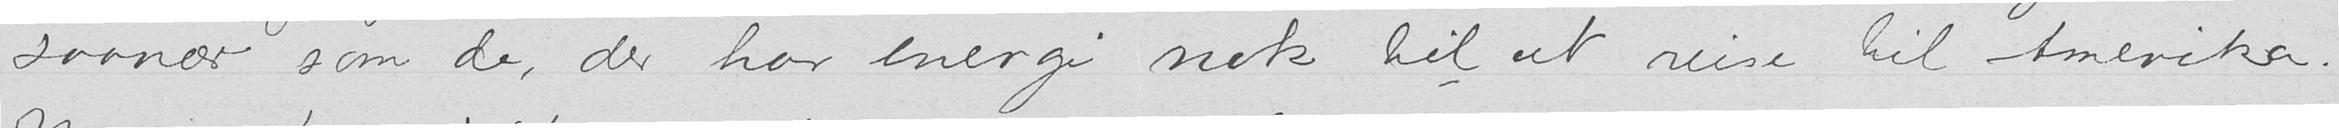saanær som de, der har energi nok til at reise til Amerika.
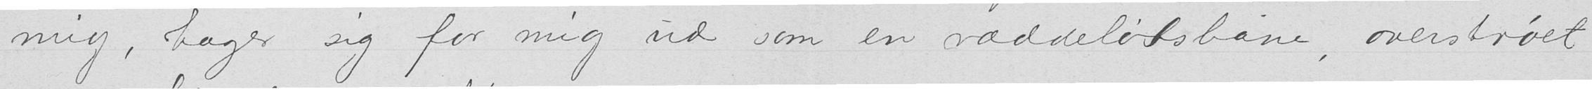mig, tager sig for mig ud som en væddeløbsbane, overstrøet
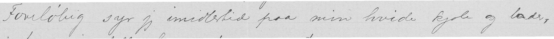Foreløbig syr jeg imidlertid paa min hvide kjole og lader,
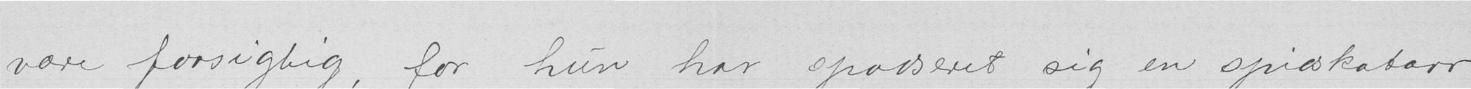være forsigtig, for hun har spadseret sig en spidskatarr
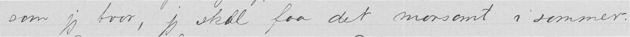som jeg tror, jeg skal faa det morsomt i sommer.
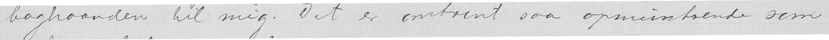baghaanden til mig. Det er omtrent saa opmuntrende som
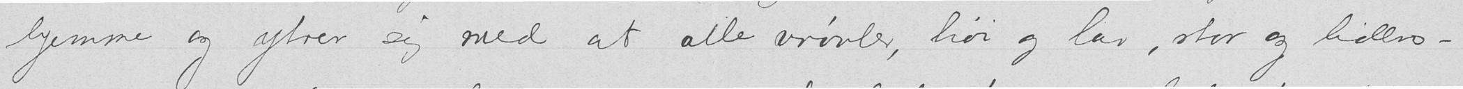hjemme og ytrer seg med at alle vrøvler, høi og lav, stor og liden -
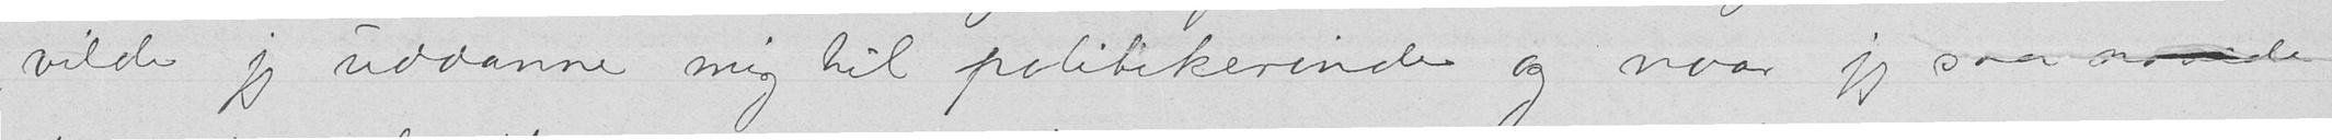vilde jeg uddanne mig til politikerinde og naar jeg saa naaede
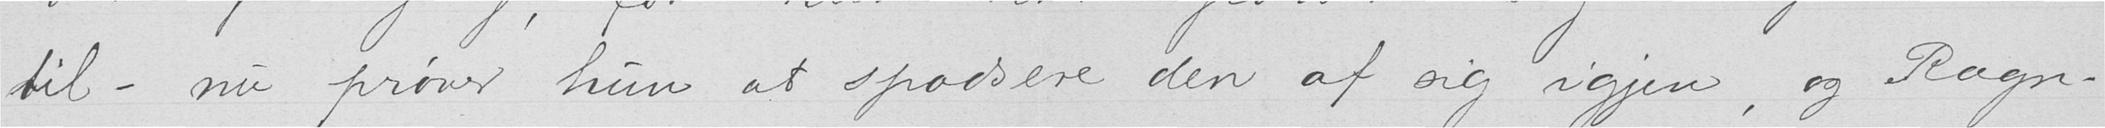til - nu prøver hun at spadsere den af sig igjen, og Ragn-
